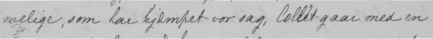melige, som har kjæmpet vor sag, Collet gaar med en
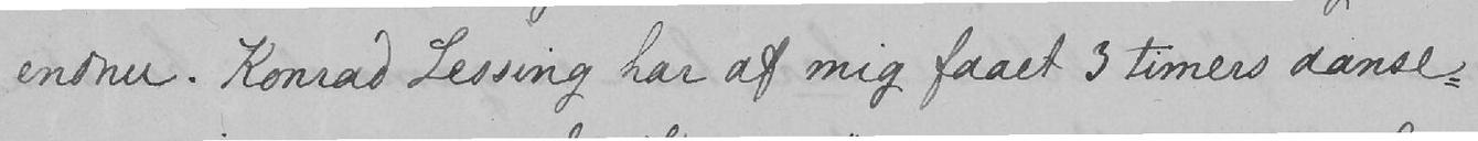endnu. Konrad Lessing har af mig faaet 3 timers danse
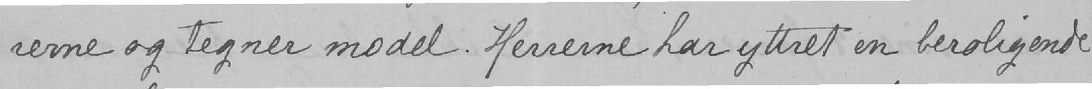rerne og tegner model. Herrerne har yttret en beroligende
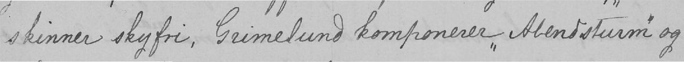skinner skyfri, Grimelund komponerer «Abendsturm» og
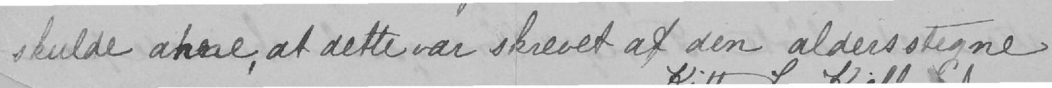skulde ahne, at dette var skrevet af den aldersstegne
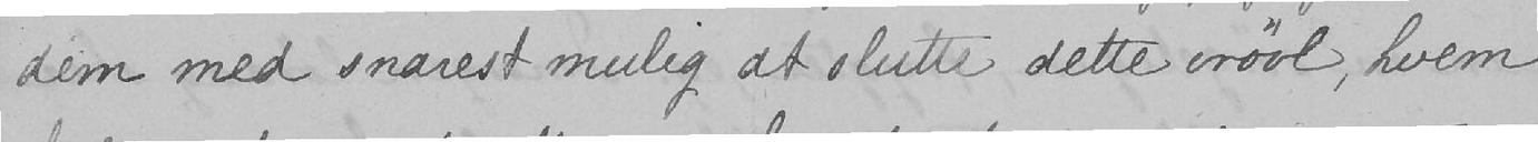dem med snarest mulig at slutte dette vrøvl, hvem
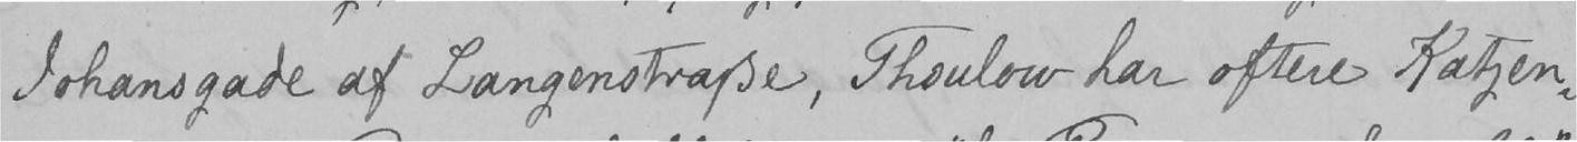Johansgade af Langenstrasse, Thoulow har oftere Katzen
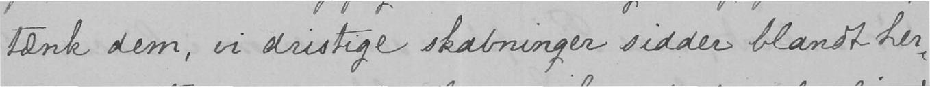tænk dem, vi dristige skabninger sidder blandt her-
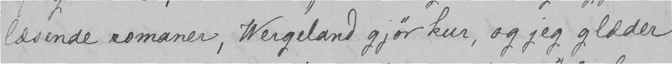læsende romaner, Wergeland gjør kur, og jeg glæder
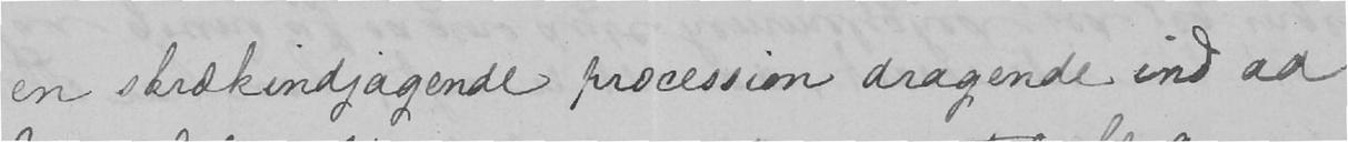en skrækindjagende procession dragende ind ad
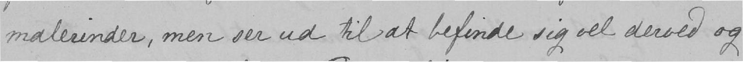malerinder, men ser ud til at befinde sig vel derved og
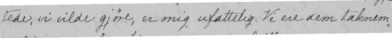tede, vi vilde gjøre, er mig ufattelig. Vi ere dem taknem-
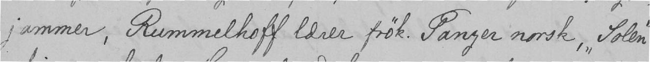jammer, Rummelhoff lærer frøk. Panzer norsk, «Solen»
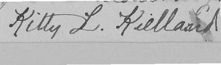Kitty L. Kielland
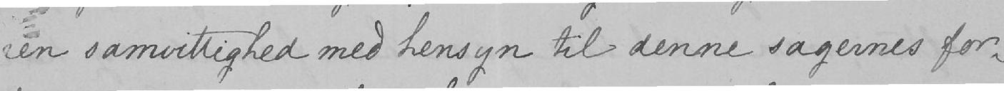ren samvittighed med hensyn til denne sagernes for-
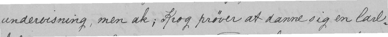undervisning, men ak; Krog prøver at danne sig en Carl-
